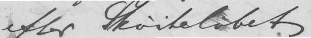efter Skøiteløbet.
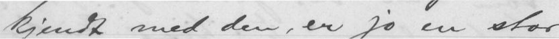kjendt med den, er jo en stor
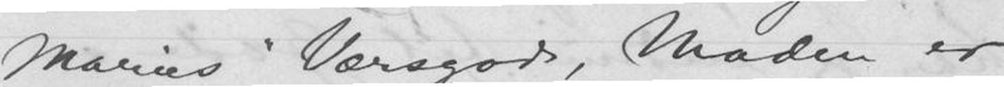Maries, "Værsgod, Maden er
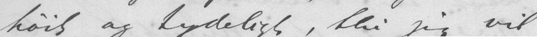høit og tydeligt, thi jeg vil
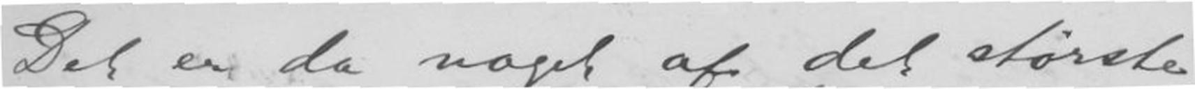Det er da noget af det største
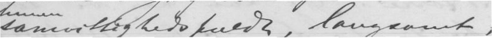samvittighedsfuldt, langsomt,
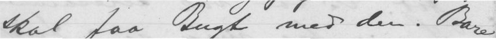skal faa Bugt med den. Bare
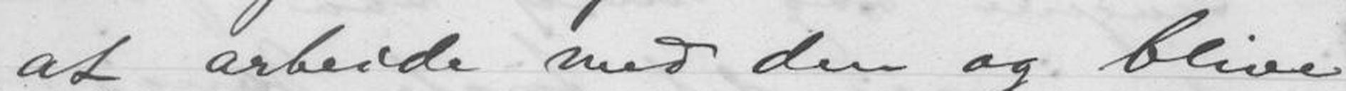at arbeide med den og blive
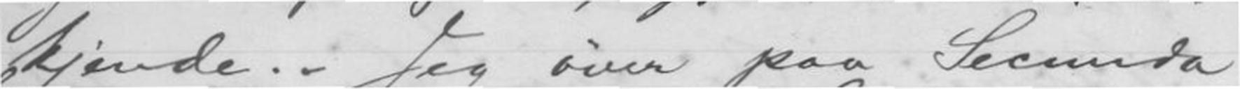kjende. - Jeg øver paa Secunda
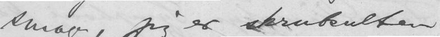smage, jeg er skrubsulten
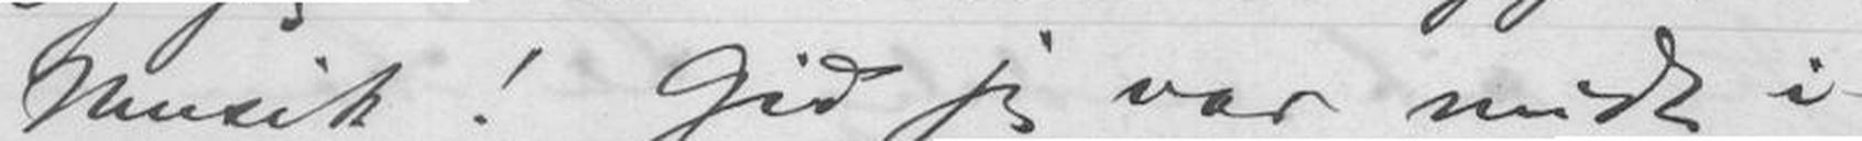Musik! Gid jeg var midt i-
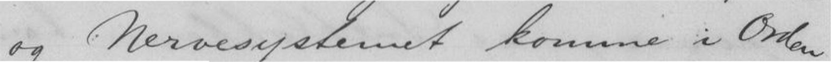og Nervesystemet komme i Orden
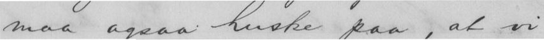maa ogsaa huske paa, at vi
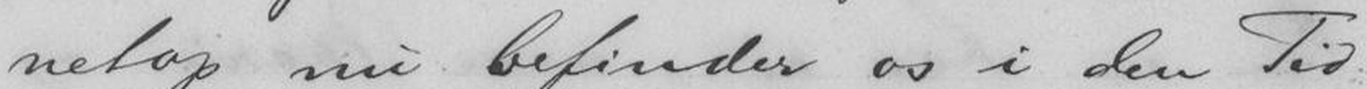netop nu befinder os i den Tid
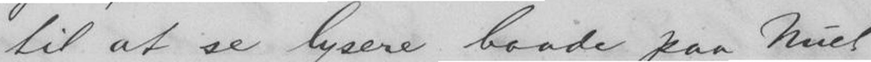til at se lysere baade paa Nuet
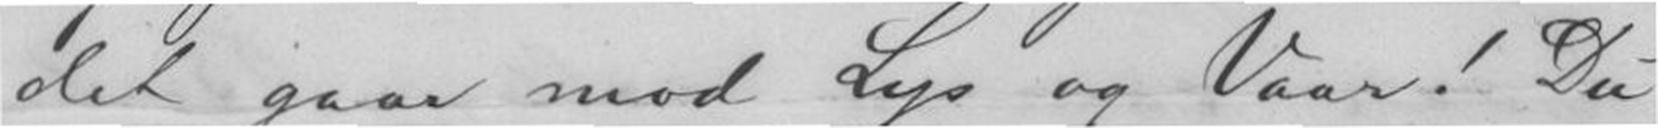det gaar mod Lys og Vaar! Du
